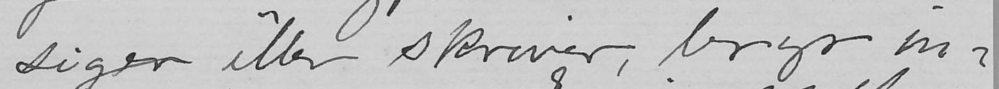siger eller skriver, bryr in-
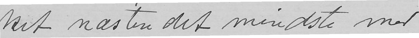ket næsten det mindste med
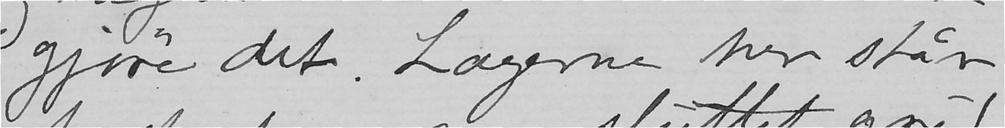gjøre det. Lægerne her står
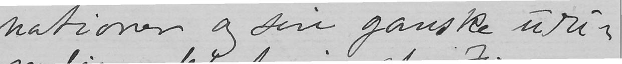nationer og sin ganske uri-
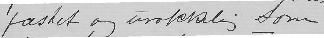fæstet og urokkelig som
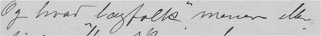Og hvad "lægfolk" mener eller
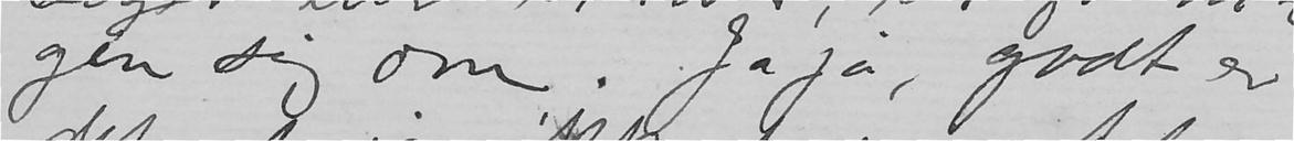gen sig om. Ja ja, godt er
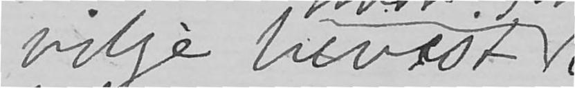vilje bevist
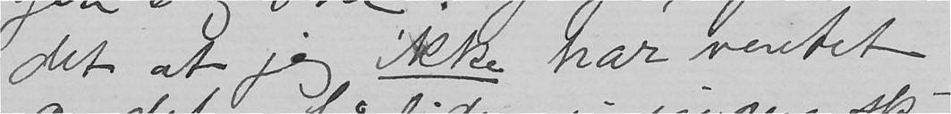det at jeg ikke har ventet
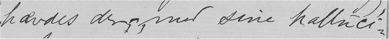hævdes der; med sine halluci-
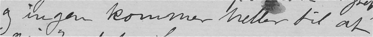og ingen kommer heller til at
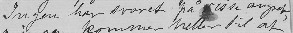Ingen har svaret på disse angreb,
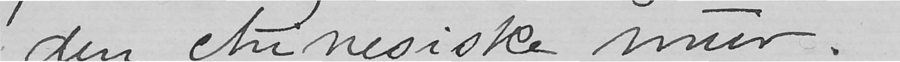den chinesiske mur.
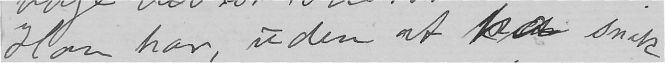Han har, uden at ha snak-
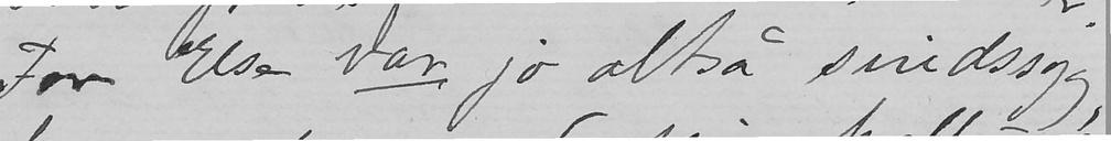For Else var jo altså sindsyg,
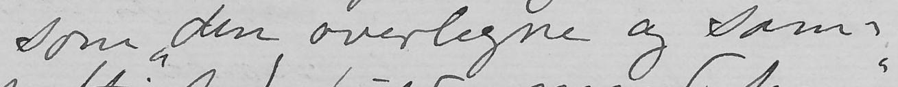som "den overlegne og sam-
